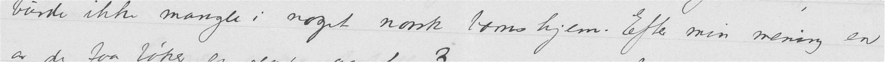burde ikke mangle i noget norsk barns hjem. Efter min mening en
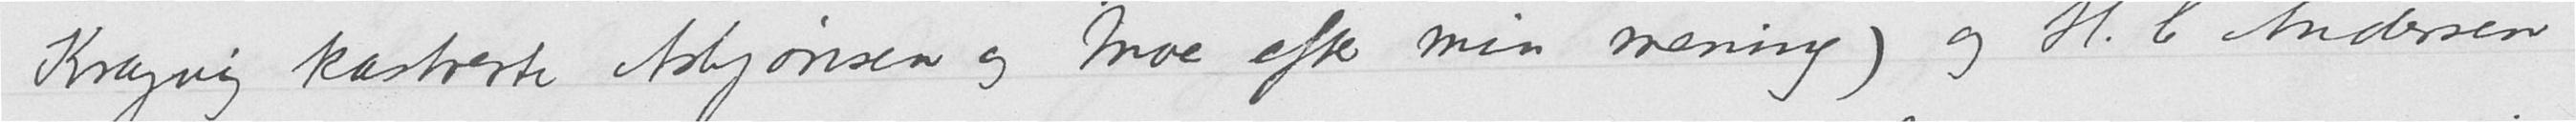Krogvig kastrerte Asbjønsen og Moe efter min mening) og H.C Andersen
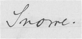Snorre.
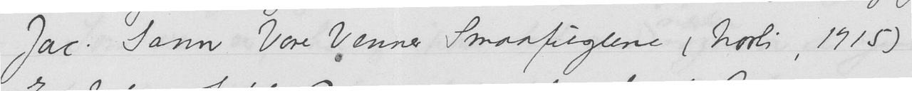Jac. Sann Vore Venner Smaafuglene (Norli, 1915)
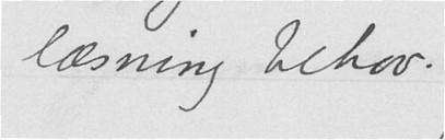læsning behov.
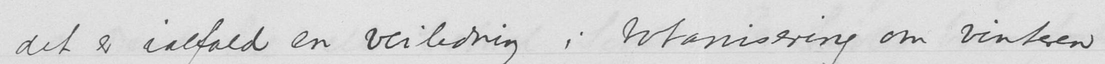det er ialfald en veiledning i botanisering om vinteren
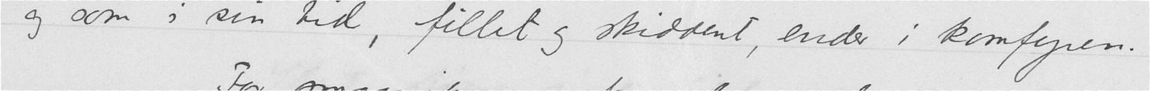og som i sin tid, fillet og skiddent, ender i komfyren.
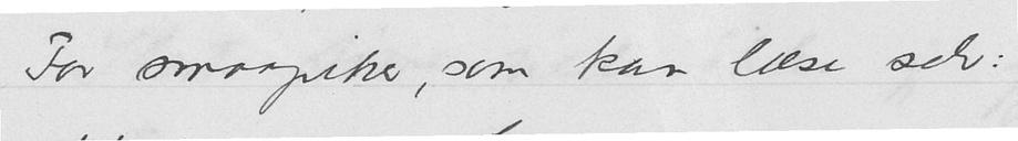For smaapiker, som kan læse selv:
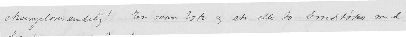eksemplarer endelig! En saan bok og en eller to lerredsbøker med
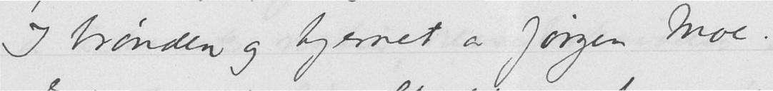I brønden og tjernet av Jørgen Moe.
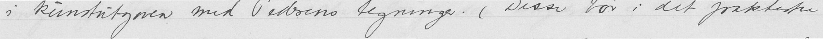i kunstutgaven med Pedersens tegninger. (Disse bør i det praktiske
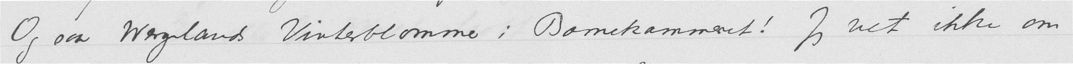Og saa Wergelands Vinterblommer i Barnekammeret! Jeg vet ikke om
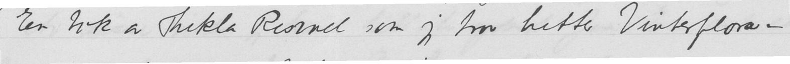En bok av Thekla Resvoll som jeg tror hetter Vinterflora –
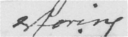erfaring
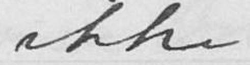ikke
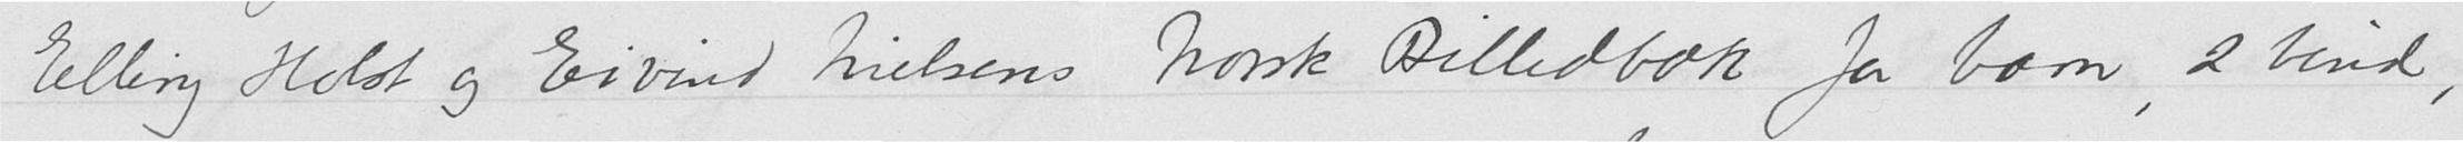Elling Holst og Eivind Nielsens Norsk Billedbok for barn, 2 bind,
